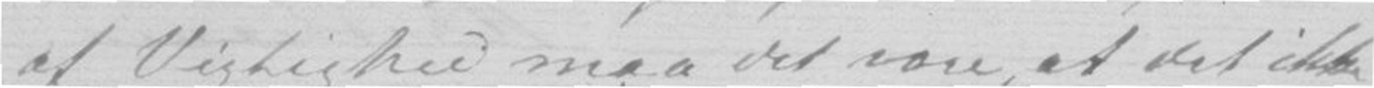af Vigtighed maa det være at det ikke
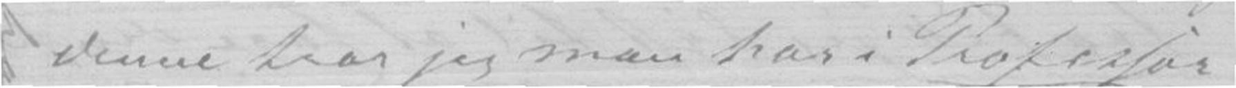denne tror jeg man har i Professor
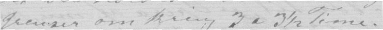Grenser omkring 3 a 3 1/2 Time. -
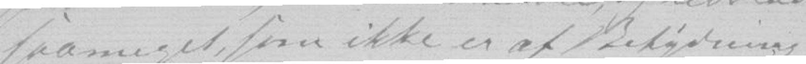saameget, som ikke er af Betydning
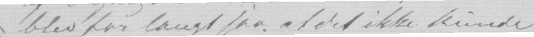blev for langt saa at det ikke kunde
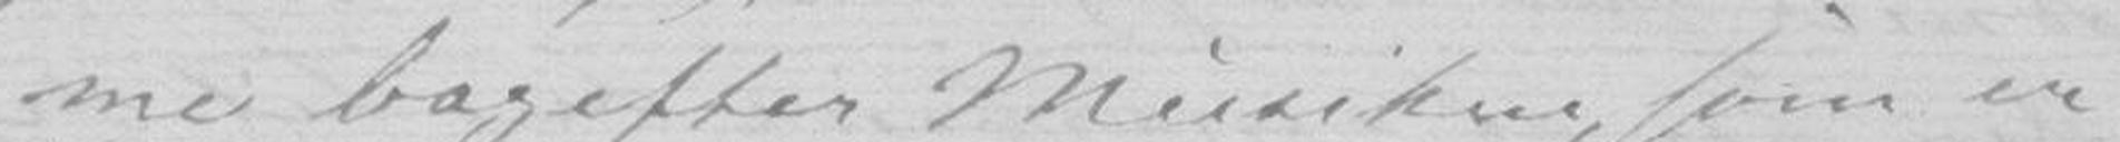me bagefter, Musiken, som er
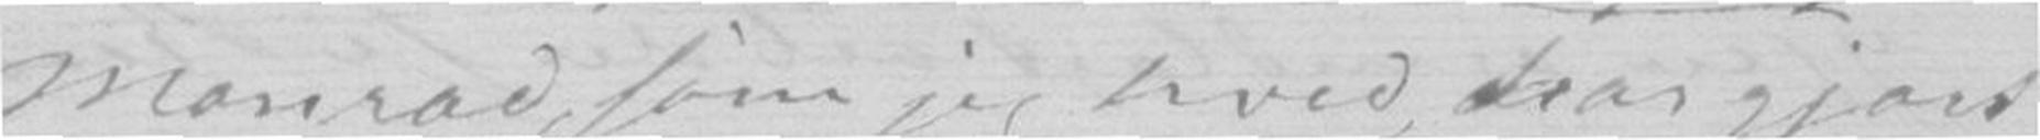Monrad, som jeg hved har gjort
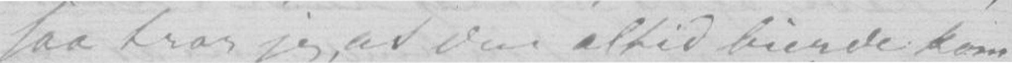saa tror jeg, at den altid burde kom-
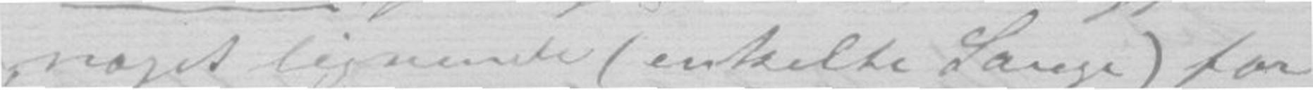noget lignende (enkelte Sange) for
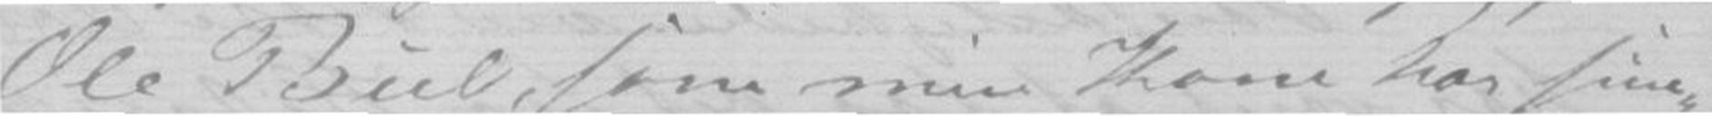Ole Bul, som min Kone har sun-
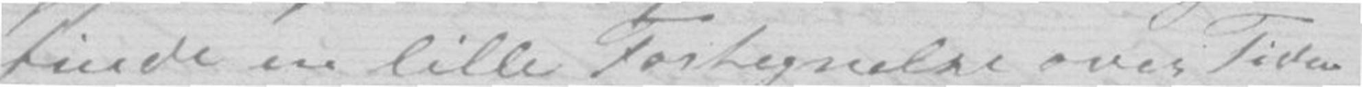finde en lille Fortegnelse over Tiden
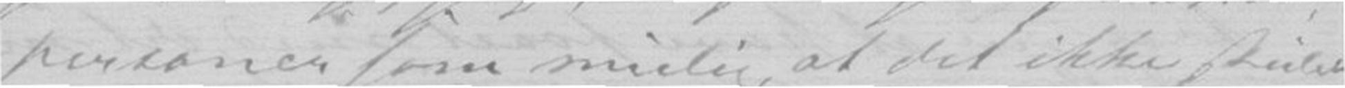personer som mulig, at det ikke skulde
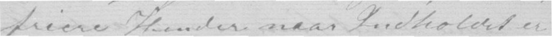friere Hender naar Indholdet er
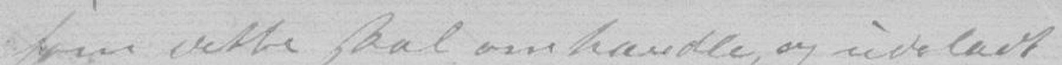som dette skal omhandle, og udeladt
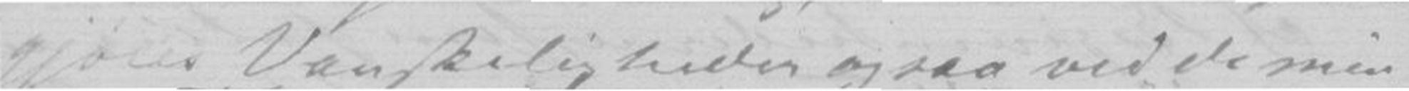gjøres Vanskeligheder ogsaa ved de min
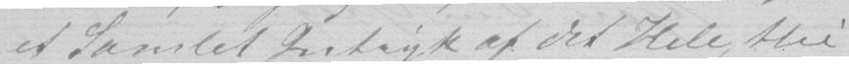et Samlet Indtryk af det Hele, thi
